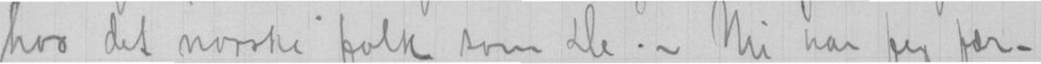hos det norske folk som de.- Nu har jeg fær-
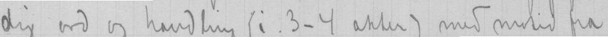dig ord og handling (i 3-4 akter) med motiv fra
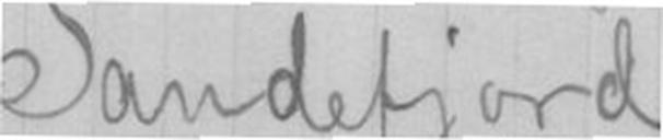Sandefjord
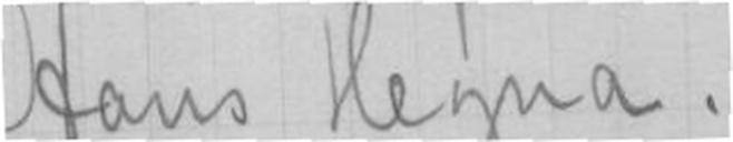Hans Hegna.
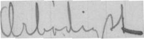Ærbødigst
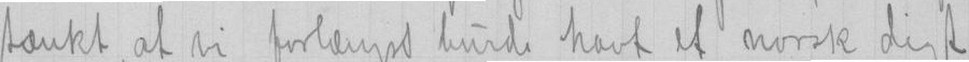tænkt, at vi forlengst burde havt et norsk digt
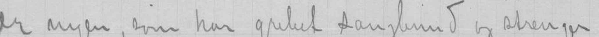er ingen, som har grebet sangbund og strenger
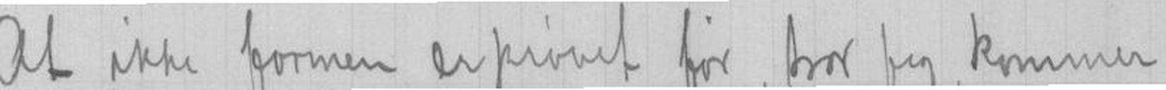At ikke formen er prøvet før, tror jeg, kommer
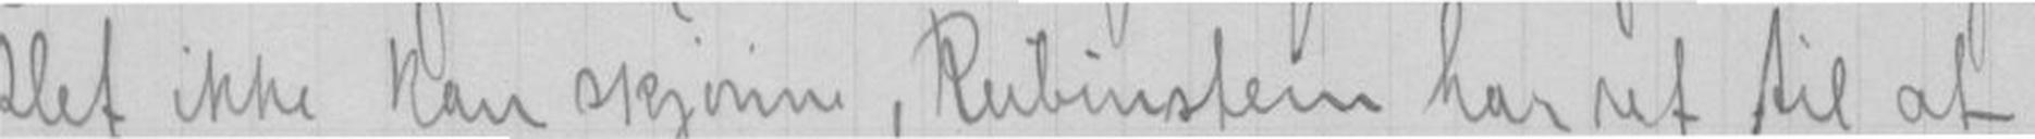slet ikke kan skjønne, Rubinstein har ret til at
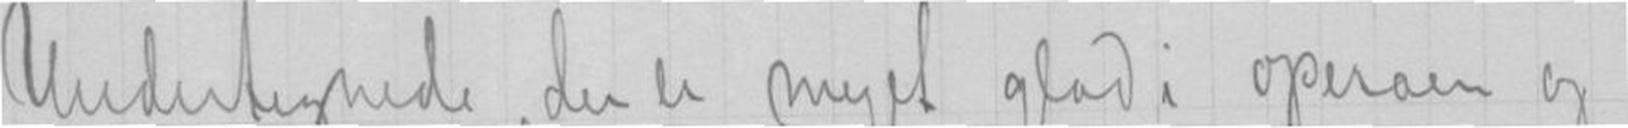Undertegnede, der er meget glad i operaen og
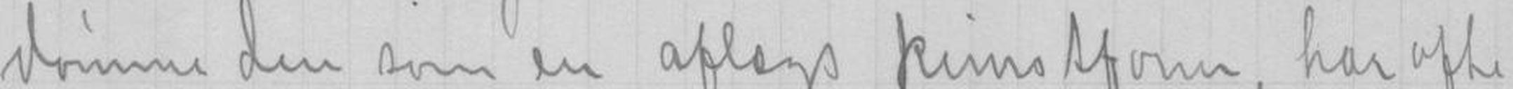dømme den som en aflegs kunstform, har ofte
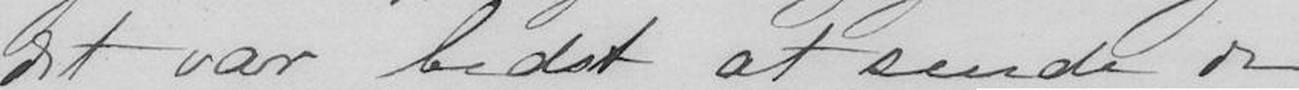det var bedst at sende de
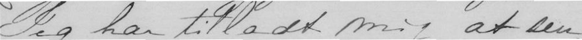Jeg har tilladt mig at sen-
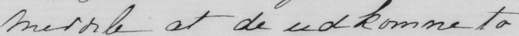meddele at de udkomne to
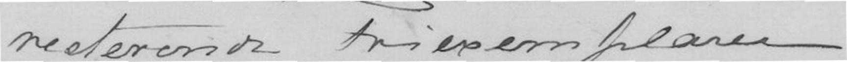resterende Friexemplarer
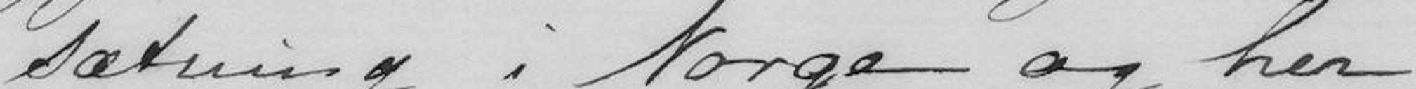sætning i Norge og her
